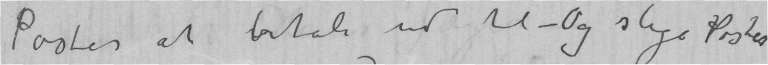Poster at betale ud til – Og slige Poster
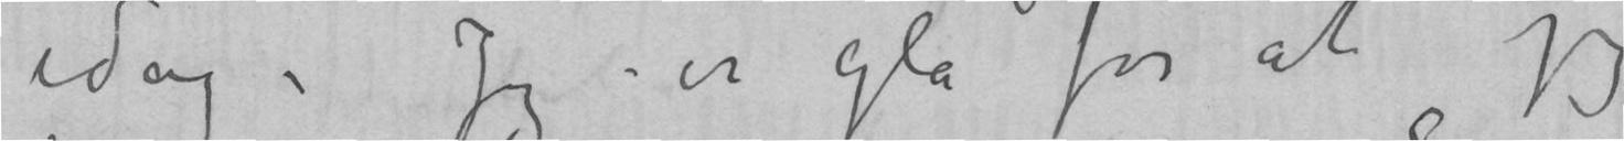idag – Jeg er gla for at jeg
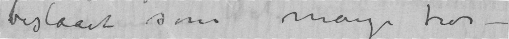beslaaet som mange tror –
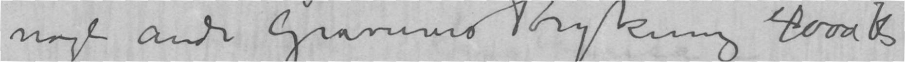nogle andre Gravurers Trykning 4000 kr
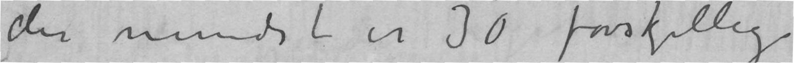der mindst er 30 forskjellige
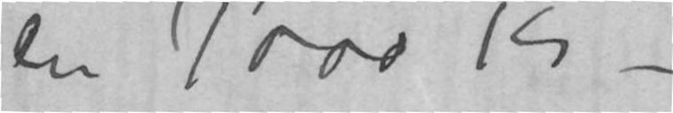en 7000 kr –
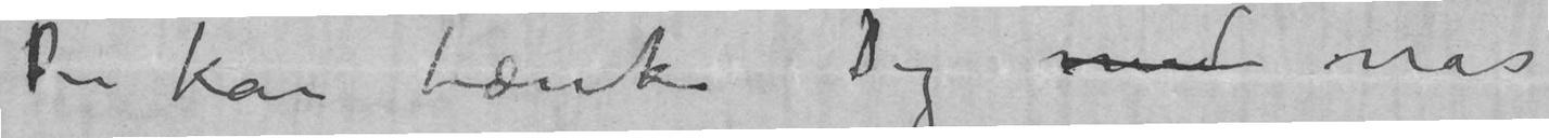Du kan tænke Dig med når
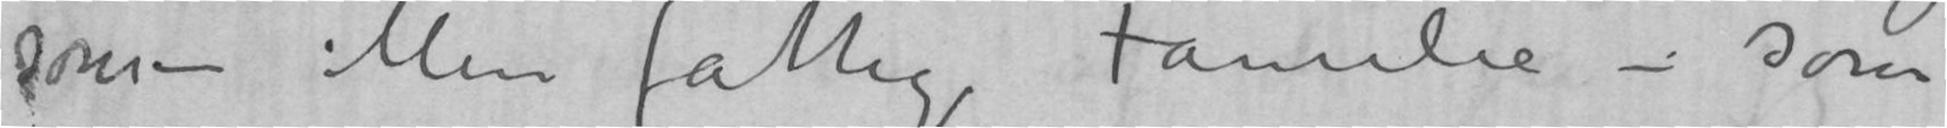som – Min fattige Familie – som
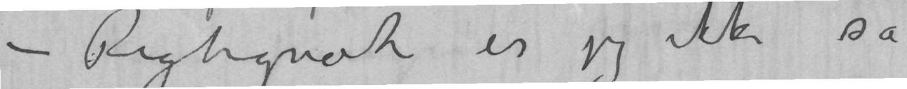– Rigtignok er jeg ikke så
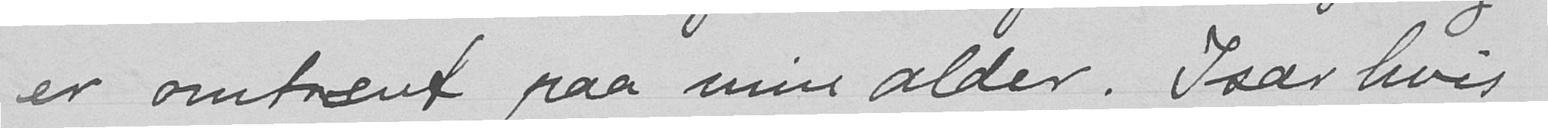er omtrent paa min alder. Især hvis
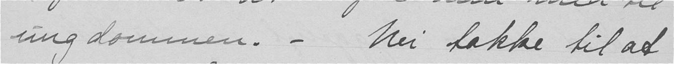ungdommen. - Nei takke til at
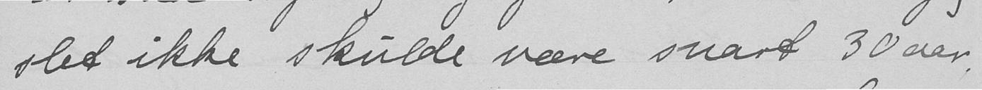slet ikke skulde være snart 30 aar.
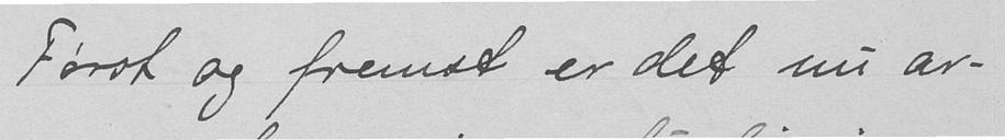Først og fremst er det nu ar-
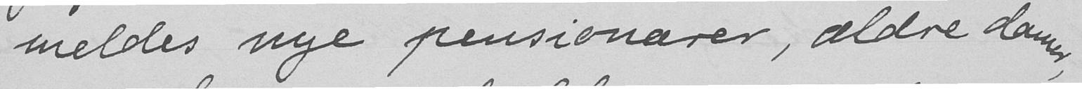meldes nye pensionærer, aldre damer
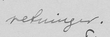retninger.
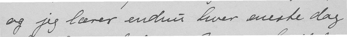og jeg lærer endnu hver eneste dag
